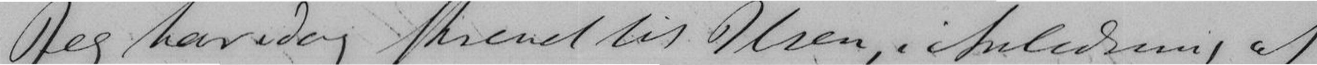Jeg har idag skrevet ti Olsen, i Anledning, af
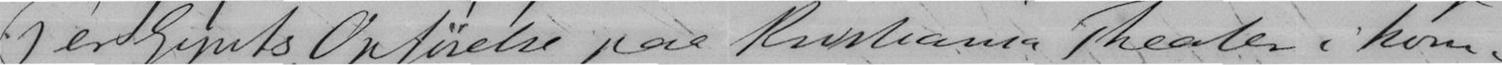Peer Gynts Opførelse paa Kunliama Theater i kom
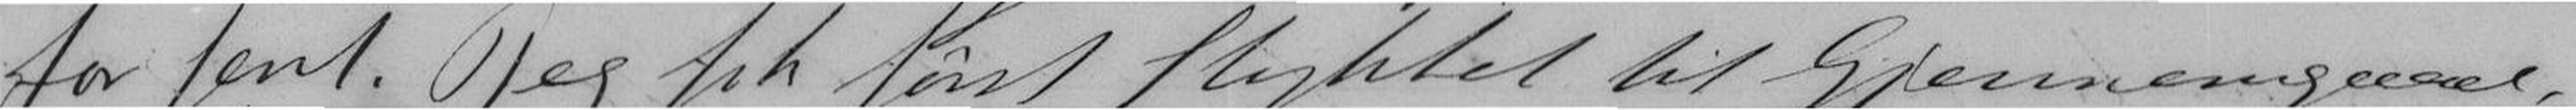for sent. Jeg fik først Stykket til Gjennemgaaæl-
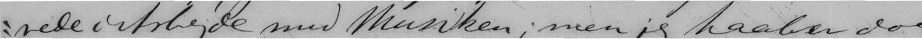rede i Arbejde med Musiken; men jeg haaber dog
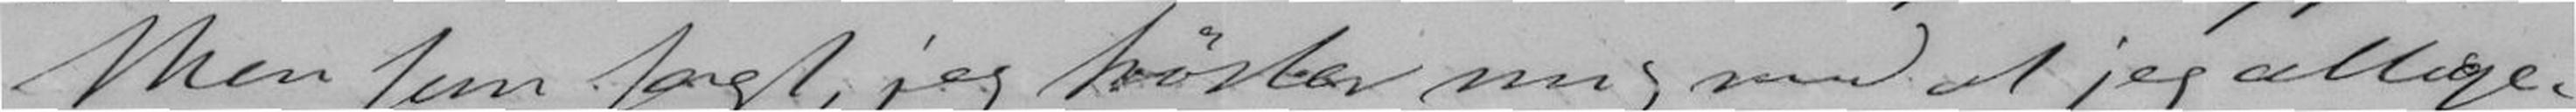Men som sagt, jeg trøster mig med at jeg allige-
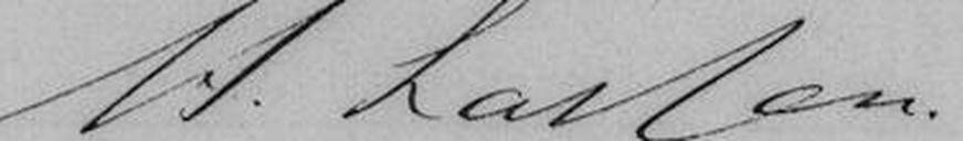M. Lassen
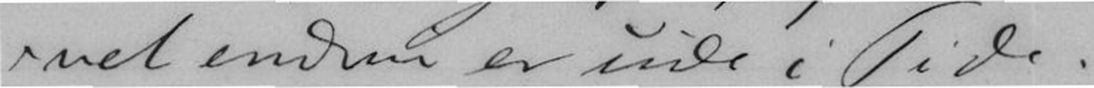vel endnu er inde i Tide.
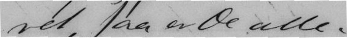ret, saa er De alle-
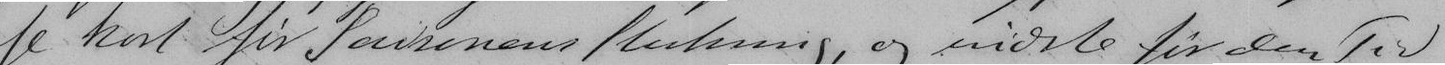se kort før Paukenens Slutning, og vidste før den Tid
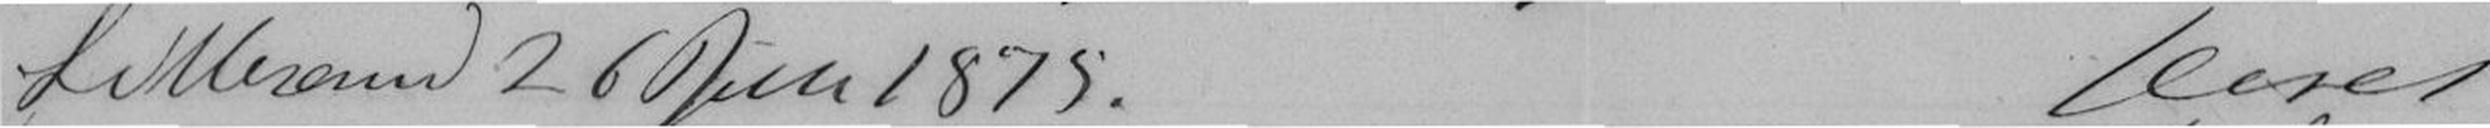Lillesand 26 Juli 1875. Deres
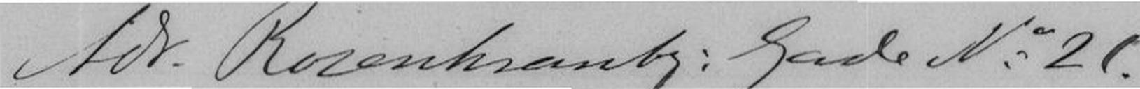Adr. Rozenkrantz: Gade No 21.
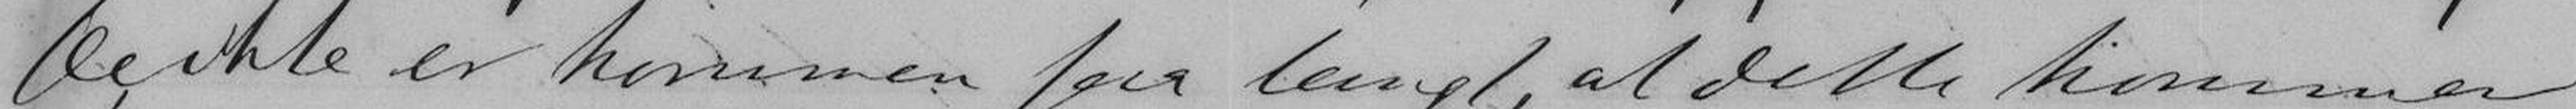De ikke er kommen saa langt, at dette kommer
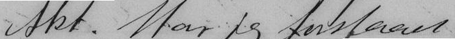Akt. Mon jeg forstaaet
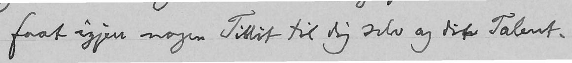faat igjen nogen Tillit til dig selv og dit Talent.
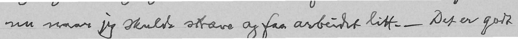nu naar jeg skulde stræve og faa arbeidet litt. - Det er godt
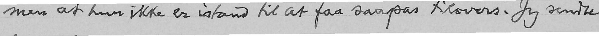men at hun ikke er istand til at faa saapas tilovers. Jeg sendte
